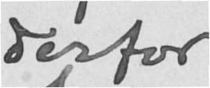derfor
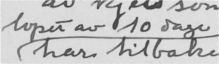lopet av 10 dage
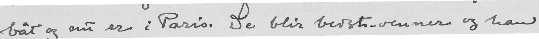båt og nu er i Paris. De blir bedste-venner og han
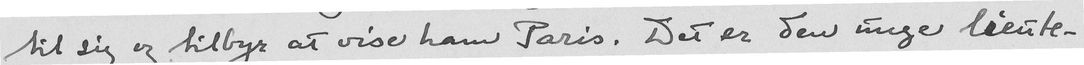til sig og tilbyr at vise ham Paris. Det er den unge lieute-
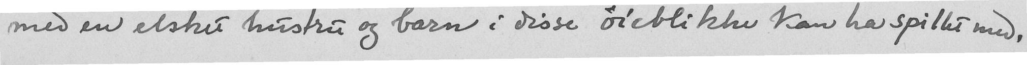med en elsket hustru og barn i disse øieblikke kan ha spillet med.
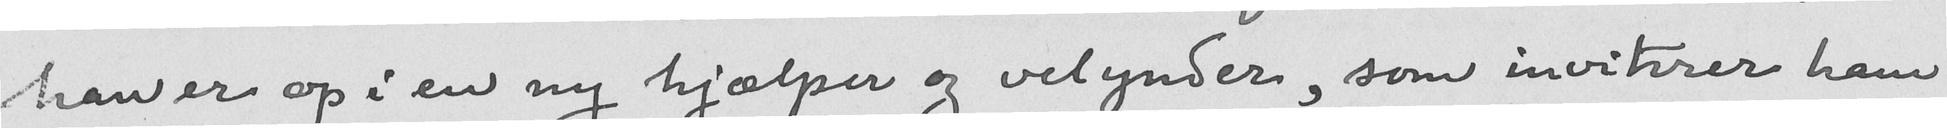han er op i en ny hjælper og velynder, som inviterer ham
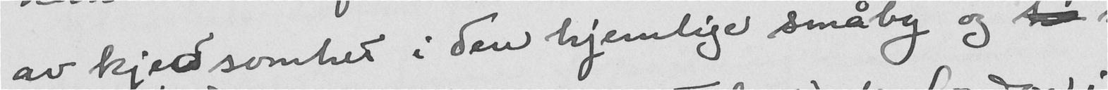av kjedsomhet i den hjemlige småby og h
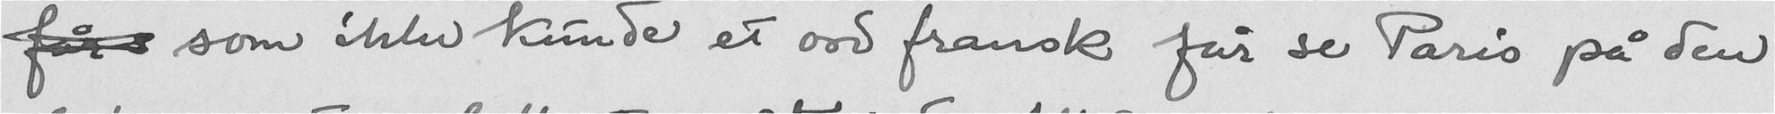får s som ikke kunde et ord fransk får se Paris på den
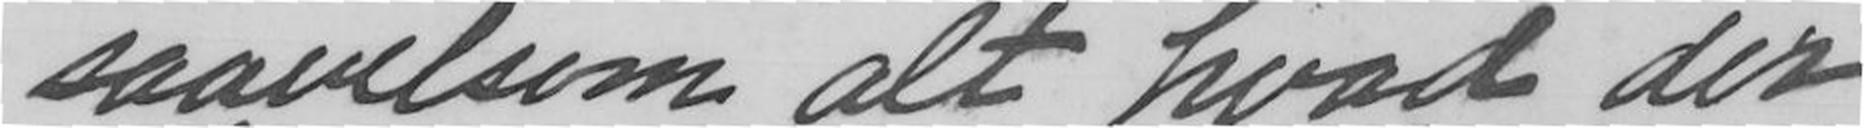saavelsom alt hvad der
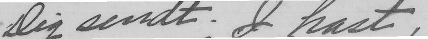Dig sendt. I hast,
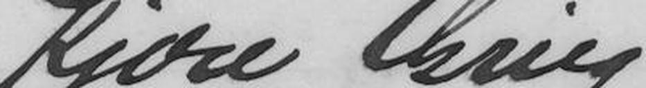Kjære Grieg!
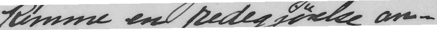komme en redegjørelse om-
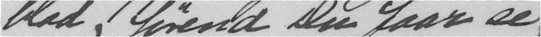blad, førend Du faar se,
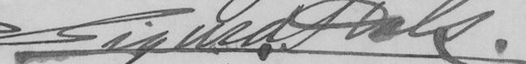Sigurd Hals.
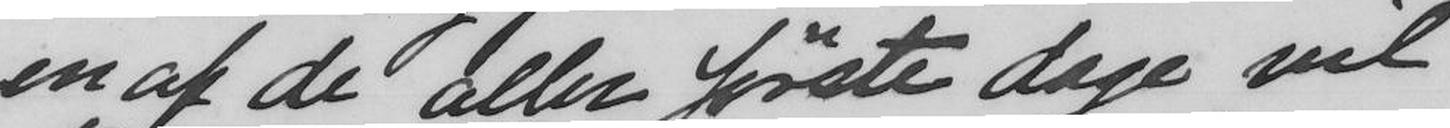en af de aller første dage vil
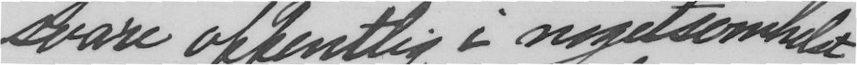svare offentlig i nogetsomhelst
